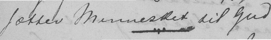sætter Mennesket til Gud.
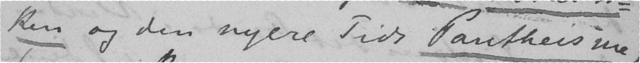ken og den nyere Tids Pantheisme)
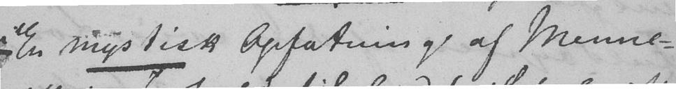En mystisk Opfatning af Menne-
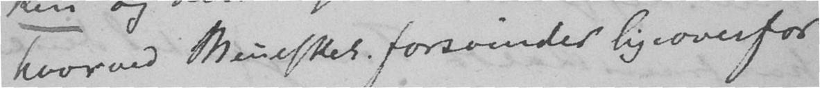hvorved Mennesket forsvinder ligeoverfor
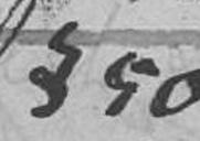§50
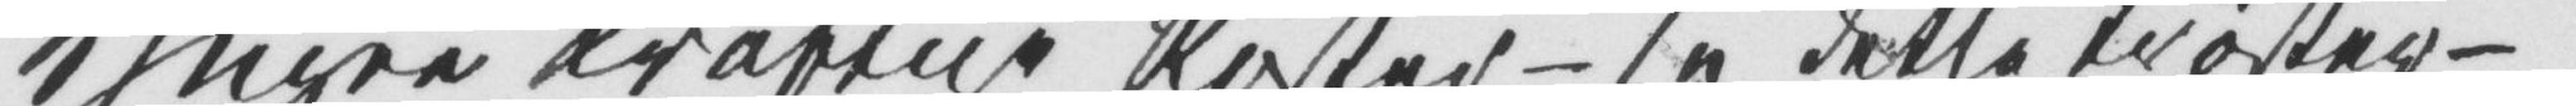Yngre kraftige Røster – se dette trøster –
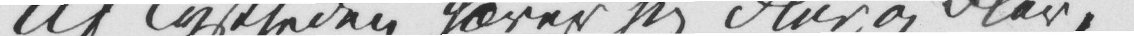Af Tystheden hæver sig Fler og Fler,
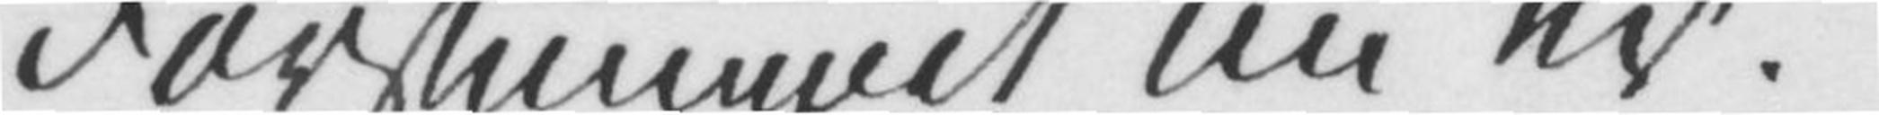Forstummet nu er.
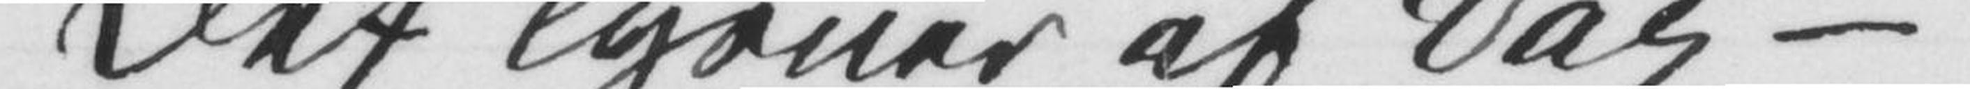Det lysner af Dag –
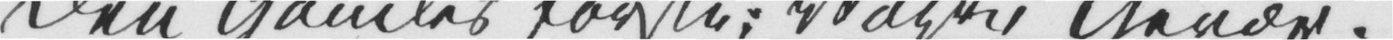Den Gamles første: Vagt i Gevær!
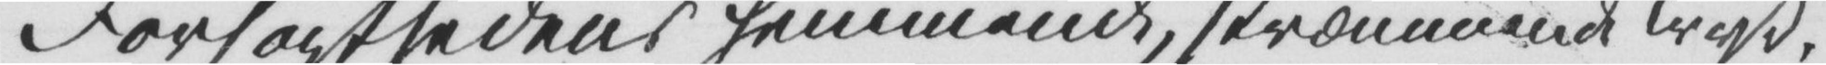Forsagthedens hemmende, skræmmende Tryk,
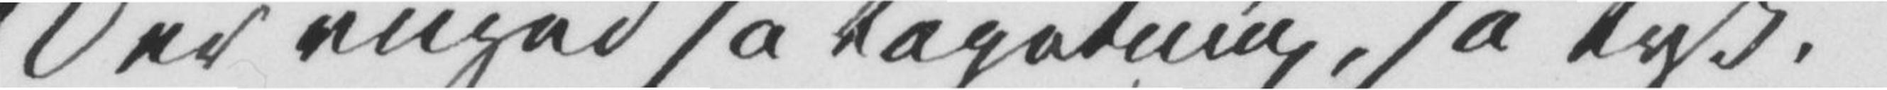Der ruged så tågetung, så tyk,
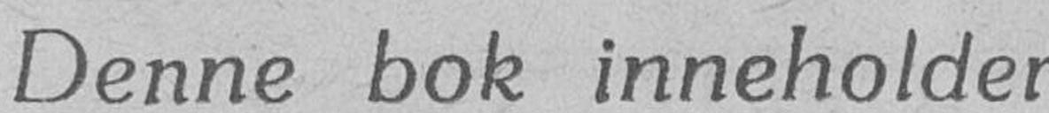Denne bok inneholder
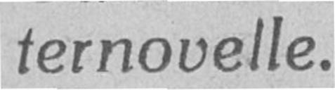ternovelle.
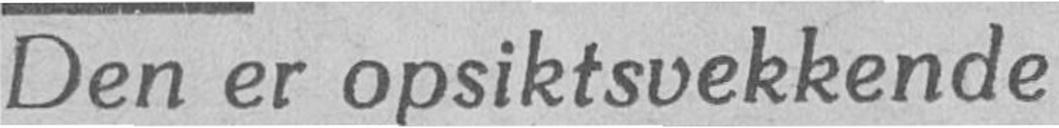Den er opsiktsvekkende
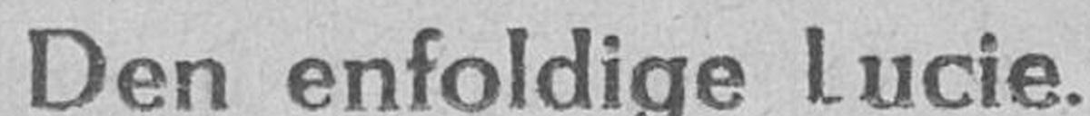Den enfoldige Lucie.
